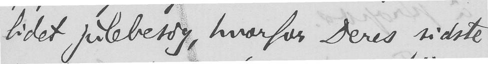lidet julebesøg, hvorfor Deres sidste
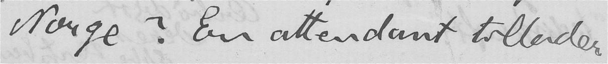Norge? En attendant tillader
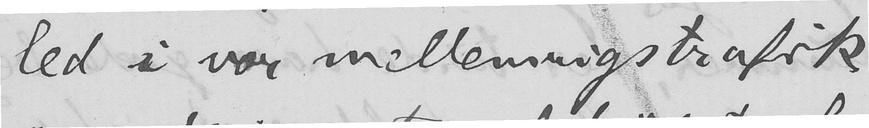led i vor mellemrigstrafik,
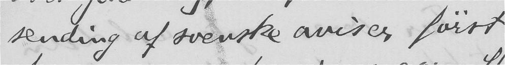sending af svenske aviser først
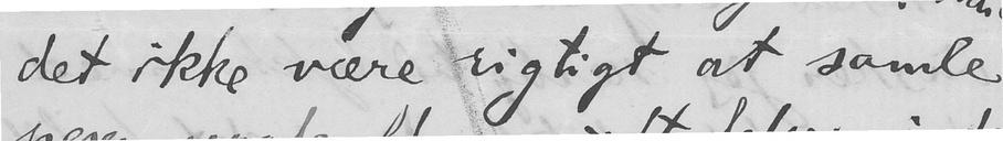det ikke være rigtigt at samle
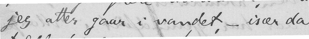jeg atter gaar i vandet, - især da
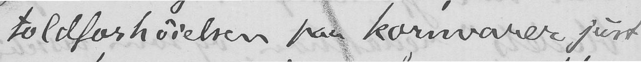toldforhøielsen paa kornvarer just
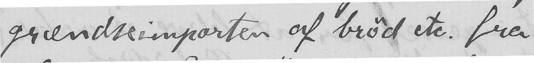grændseimporten af brød etc. fra
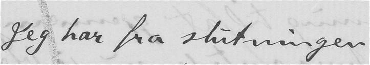Jeg har fra slutningen
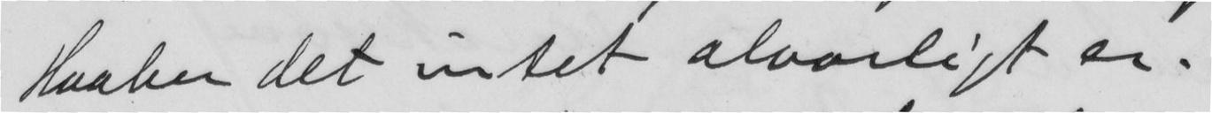Haaber det intet alvorligt er.
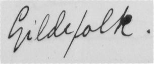Gildefolk.
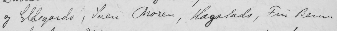og Eldegards, Sven Moren, Hægstads, Fru Berner
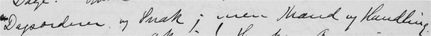Dagsordner og Snak; men Mand og Handling.
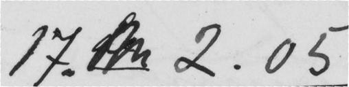17. 2.05
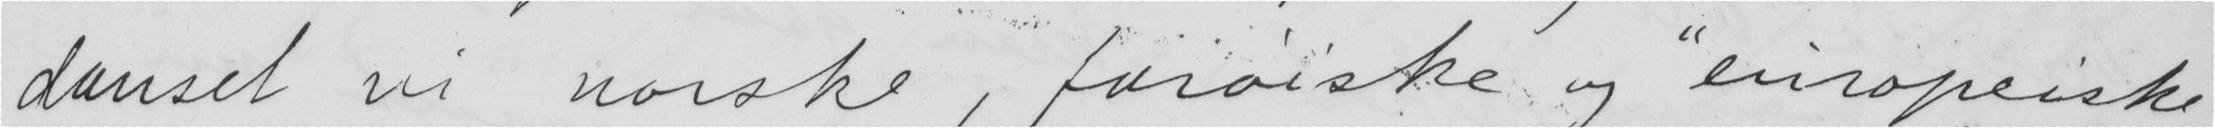danset vi norske, færøiske og "europeiske
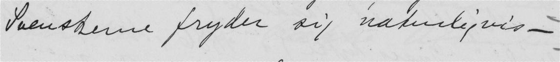Svenskerne fryder sig naturligvis -
